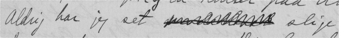Aldrig har jeg set  slige
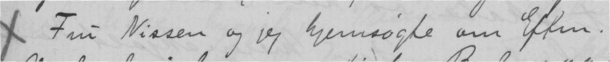x Fru Nissen og jeg hjemsøgte om eftm.
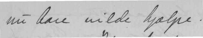nu bare vilde hjælpe.
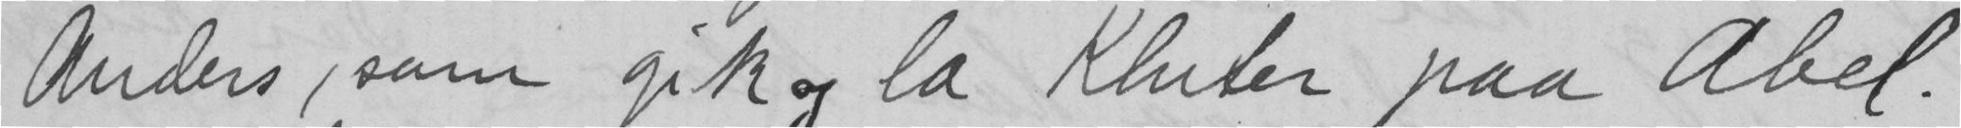Anders, som gik og la Kluter paa Abel.
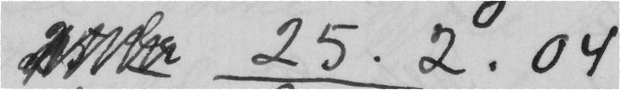25.2.04
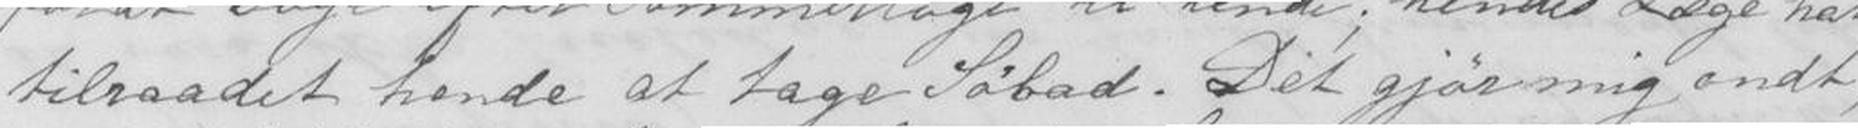tilraadet hende at tage Søbad. Det gjør mig ondt,
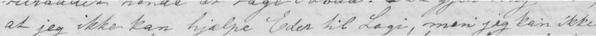at jeg ikke kan hjælpe Eder til Logi, men jeg kan ikke
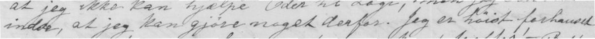indse, at jeg kan gjøre noget derfor. Jeg er høist forbauset
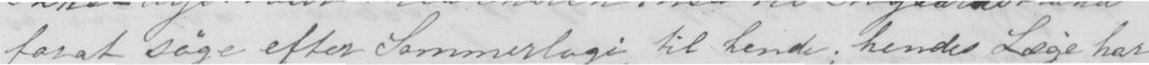forat søge efter Sommerlogi til hende; hends Læge har
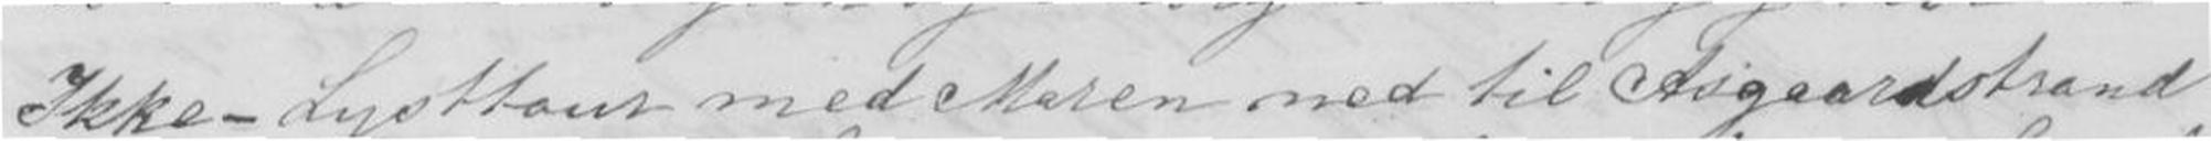Ikke-Lysttour med Maren ned til Asgaardstrand
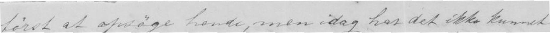først at spørge hende, men idag har det ikke kunnet
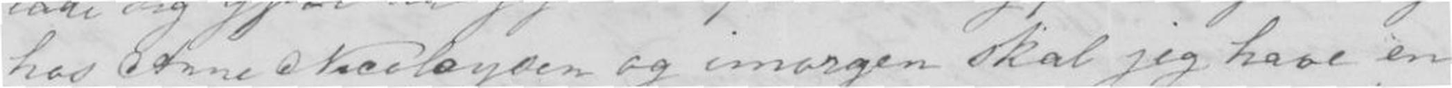hos Anne Nicolaysen og imorgen skal jeg have en
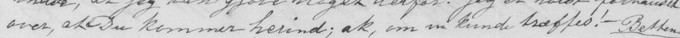over, at Du kommer herind; ak, om nu vi kunde træffes!- Betten
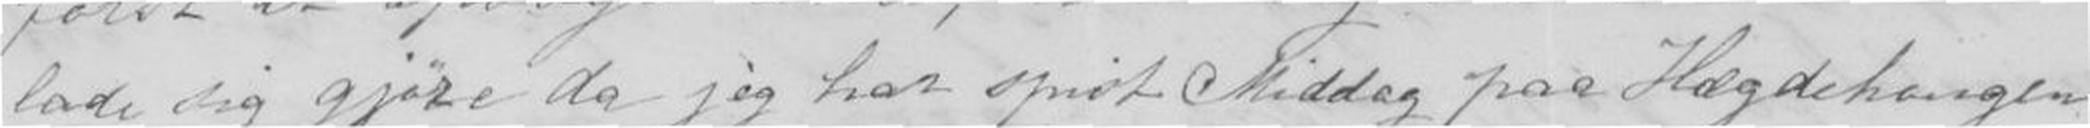lade sig gjøre da jeg har spist Middag paa Hægdetangen
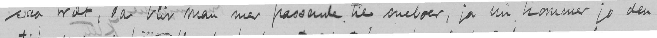saa træt, da blir man mer passende til eneboer, ja nu kommer jo den
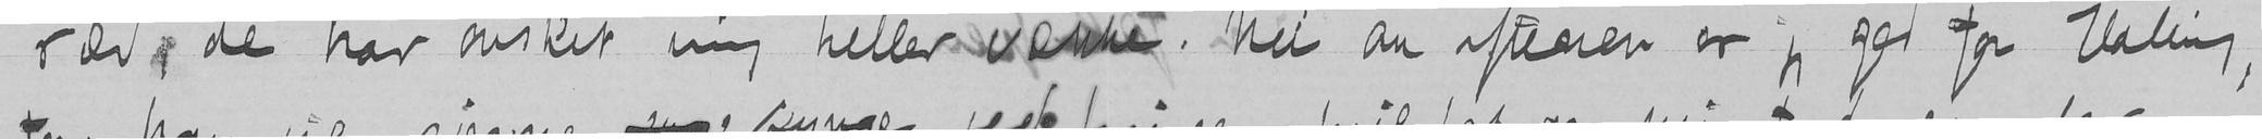ræd, de har ønsket mig heller vekke. Nu om aftenen er jeg god for Halling,
